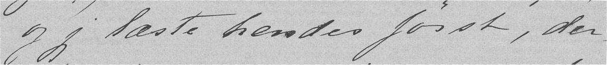og jeg læste hendes først, der-
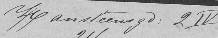Hansteensgd. 2 IV
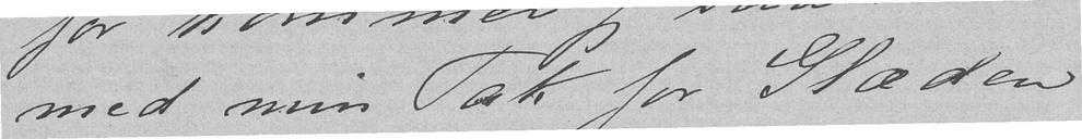med min Tak for Glæden
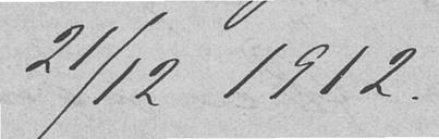21/12 1912.
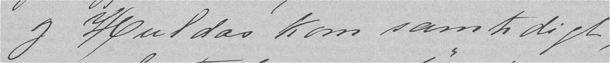og Huldas kom samtidigt,
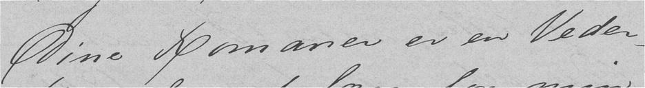Dine Romaner er en Veder-
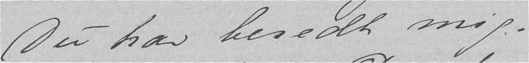Du har beredt mig.
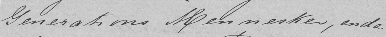Generations Mennesker, enda
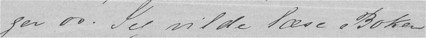ger os. Jeg vilde læse Boken
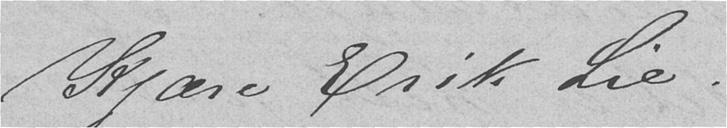Kjære Erik Lie.
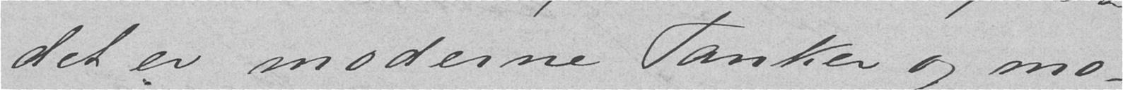det er moderne Tanker og mo-
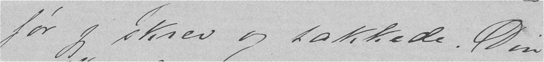før jeg skrev og takkede. Din
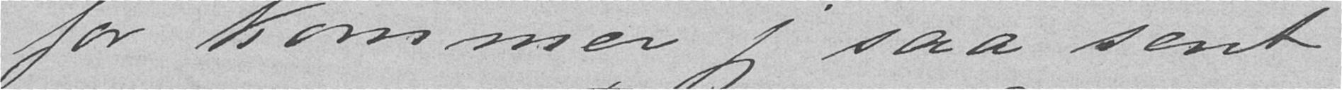for kommer jeg saa sent
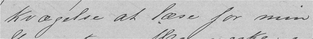kvægelse at læse for min
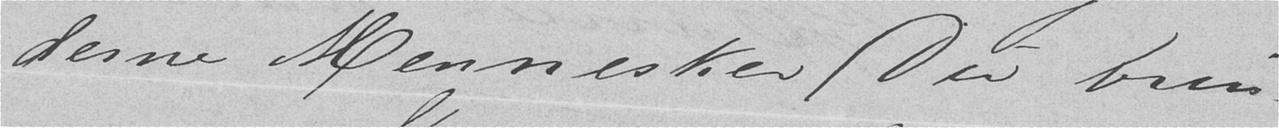derne Mennesker Du brin-
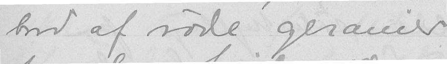baad af røde geranier
